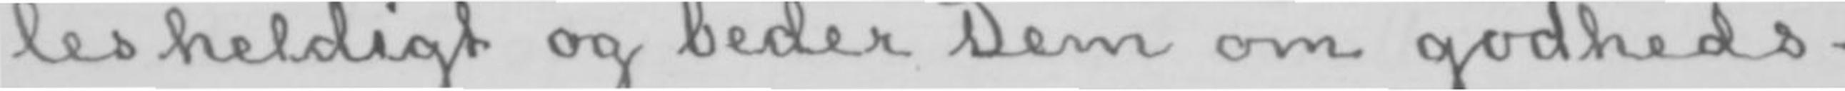les heldigt og beder Dem om godheds-
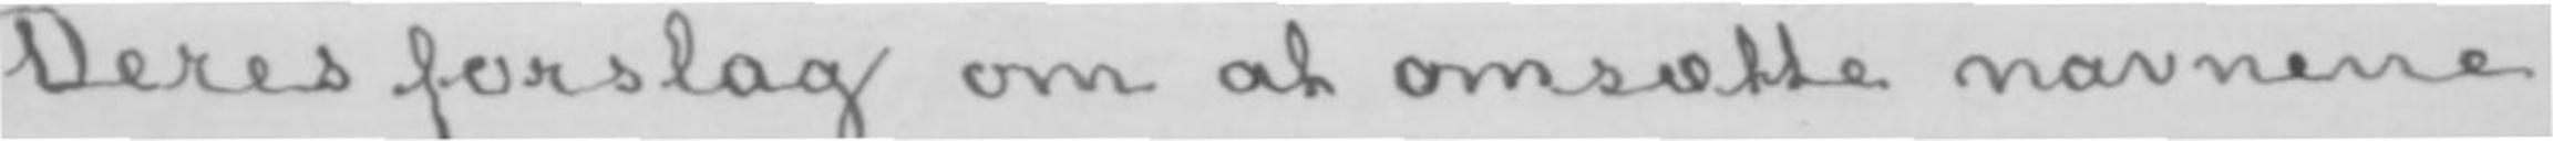Deres forslag om at omsætte navnene
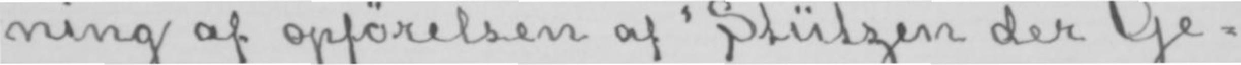ning af opførelsen af «Stützen der Ge-
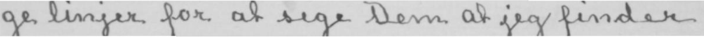ge linjer for at sige Dem at jeg finder
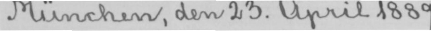München, den 23. April 1889.
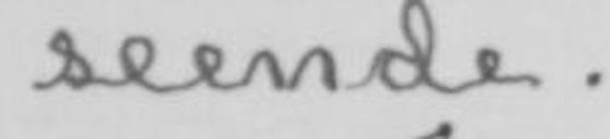seende.
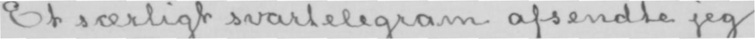Et særligt svartelegram afsendte jeg
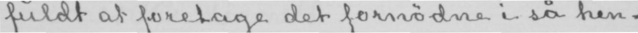fuldt at foretage det fornødne i så hen-
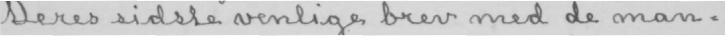Deres sidste venlige brev med de man-
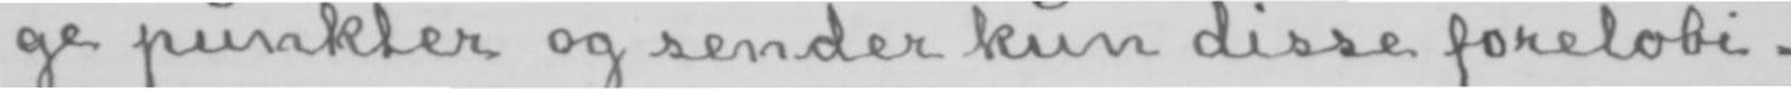ge punkter og sender kun disse foreløbi-
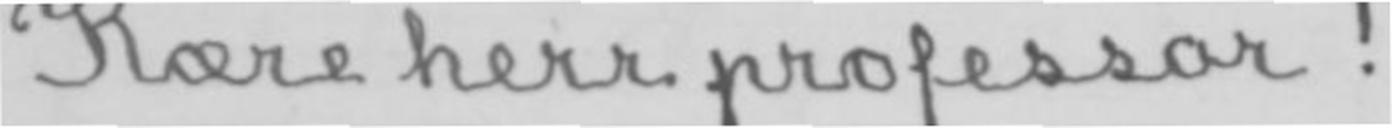Kære herr professor!
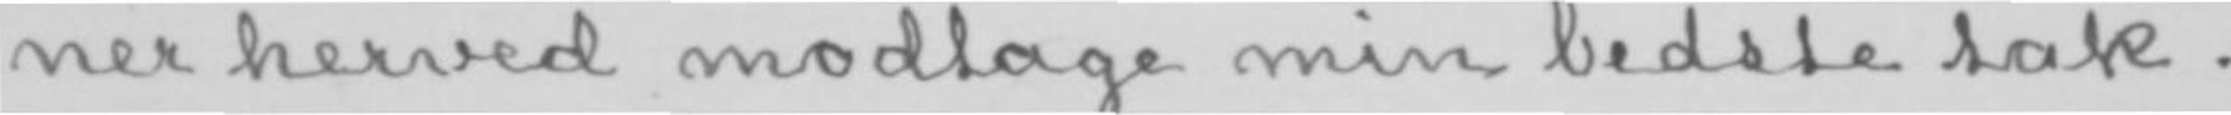ner herved modtage min bedste tak.
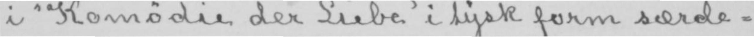i «Komödie der Liebe» i tysk form særde-
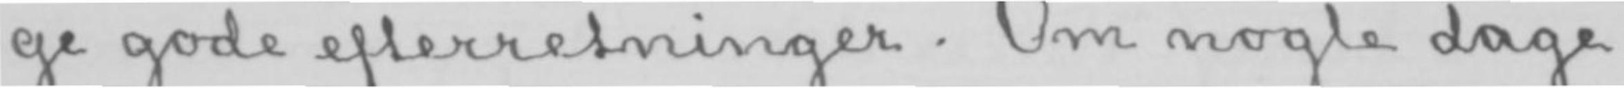ge gode efterretninger. Om nogle dage
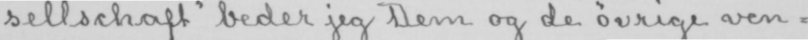sellschaft» beder jeg Dem og de øvrige ven-
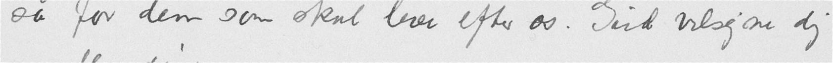så for dem som skal leve efter os. Gud velsigne dig
